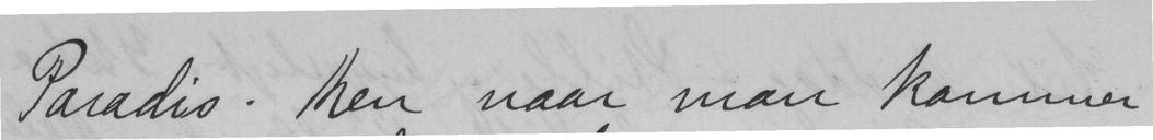Paradis. Men naar man kommer
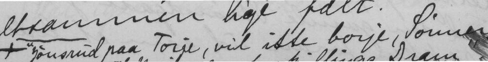- Jonsrud paa Torje, vil itte borje, Sønner
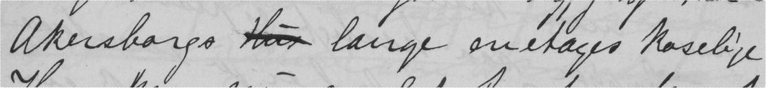Akersborgs Hus lange enetages koselige
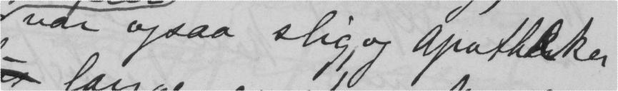var ogsaa slig og Apotheiker
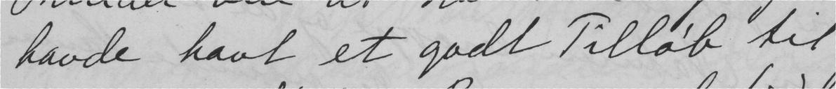havde havt et godt Tilløb til
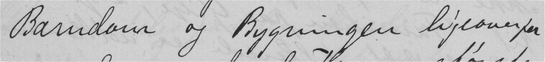Barndom og Bygningen ligeoverfer
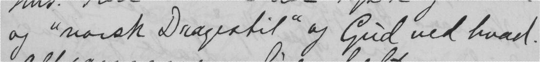og "norsk Dragestil" og Gud ved hvad.
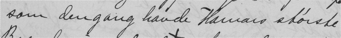som dengang havde Hamars største
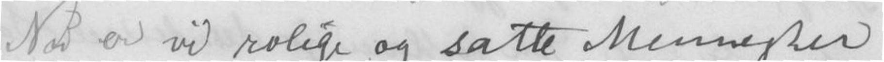Nu er vi rolige og satte Mennesker
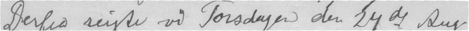Derfra reiste vi Torsdagen den 24de Aug.
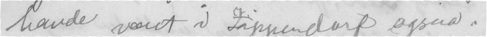havde været i Zippendorf ogsaa.
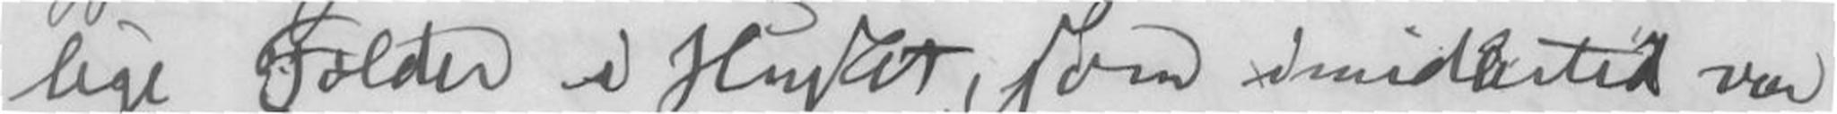lige Folder i Huset, som imidlertid vor
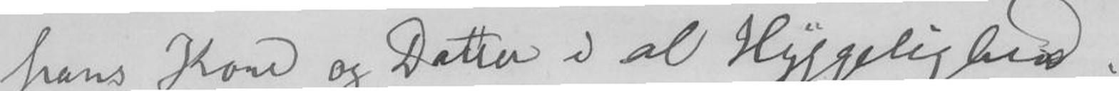hans Kone og Datter i al Hyggelighed.
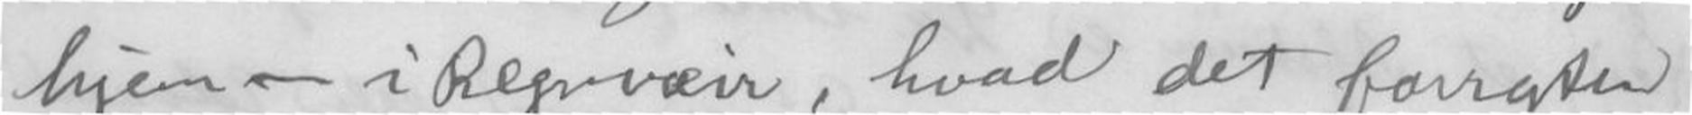hjem - i Regnveir, hvad det forresten
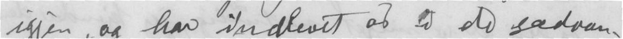igjen, og har indlevet os i de sædvan-
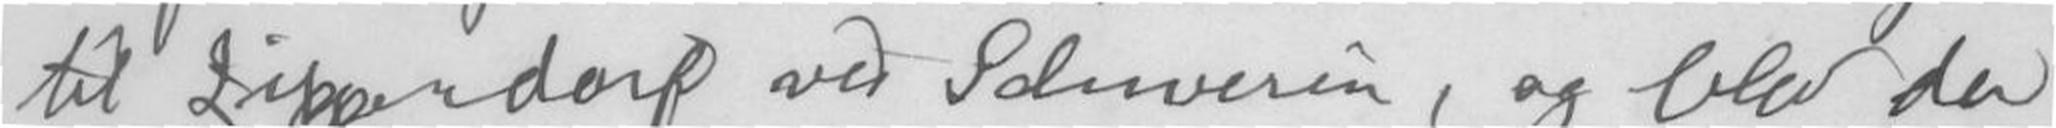til Zippendorf ved Schwerin, og blev der
